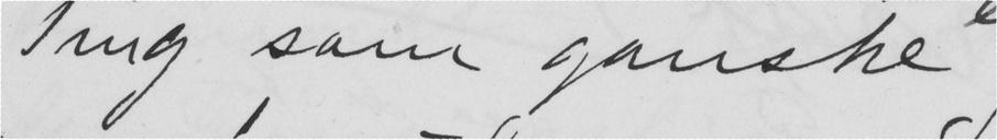Ting som ganske
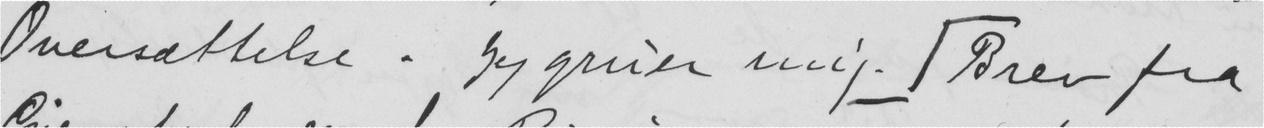Oversættelse. Jeg gruer mig. Brev fra
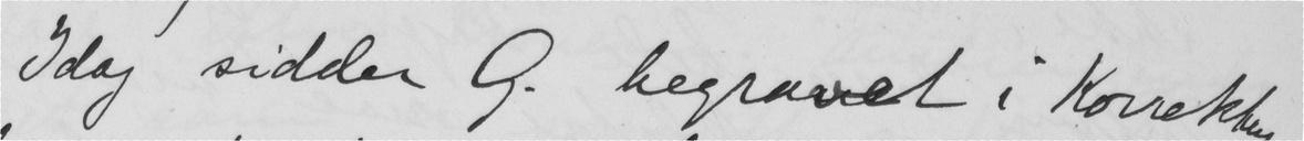Idag sidder G. begravet i Korrektur
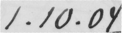1.10.04
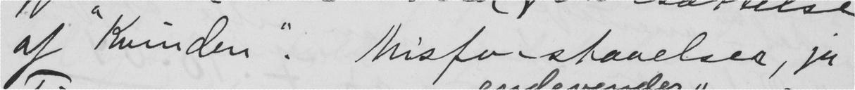af "Kvinden". Misforstaaelser, ja
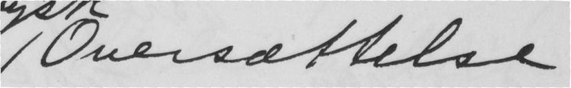Oversættelse
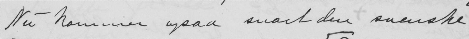Nu kommer ogsaa snart den svenske
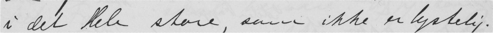i det Hele store, som ikke er lystelig.
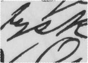tysk
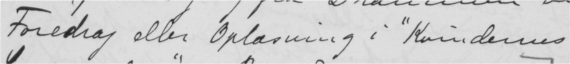Foredrag eller Oplæsning i "Kvindernes
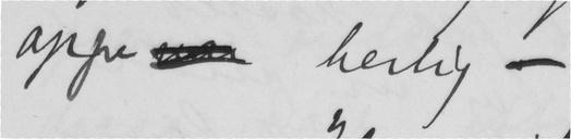oppe  herlig —
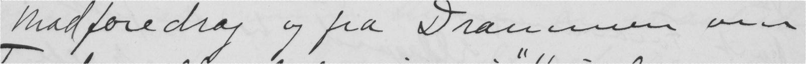Madforedrag og fra Drammen om
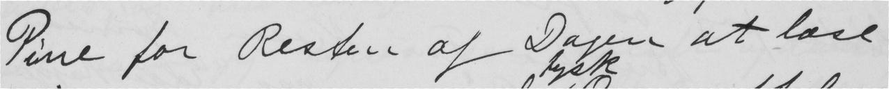Pine for Resten af Dagen at læse
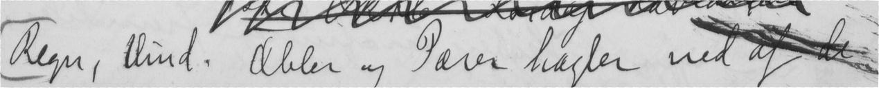Regn, Vind. Æbler og Pærer hagler ned af de
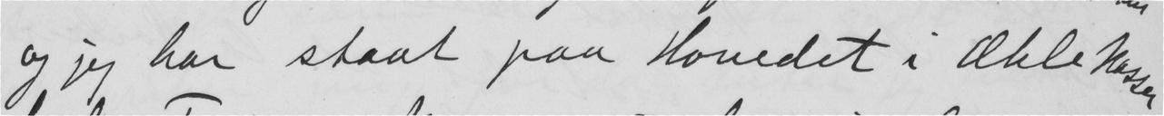og jeg har staat paa Hovedet i Æblekasser
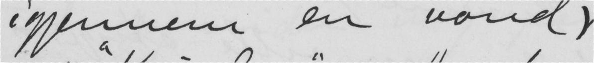igjennem en vond
